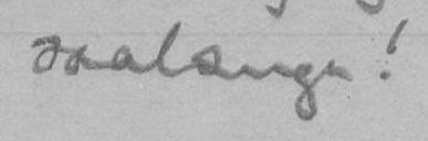saalænge!
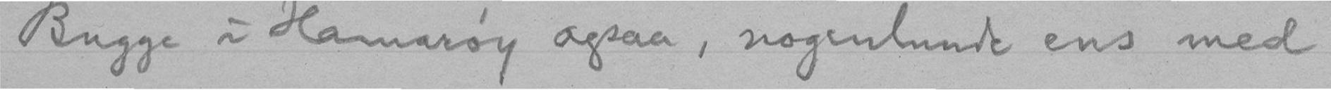Bugge i Hamarøy ogsaa, nogenlunde ens med
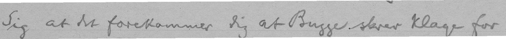Sig at det forekommer dig at Bugge skrev Klage for
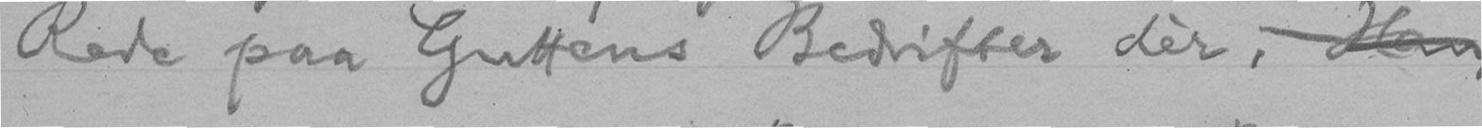Rede paa Guttens Bedrifter dèr, Han
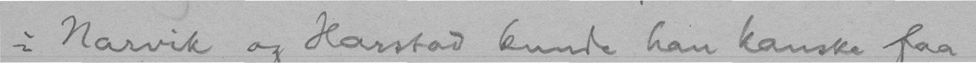i Narvik og Harstad kunde han kanske faa
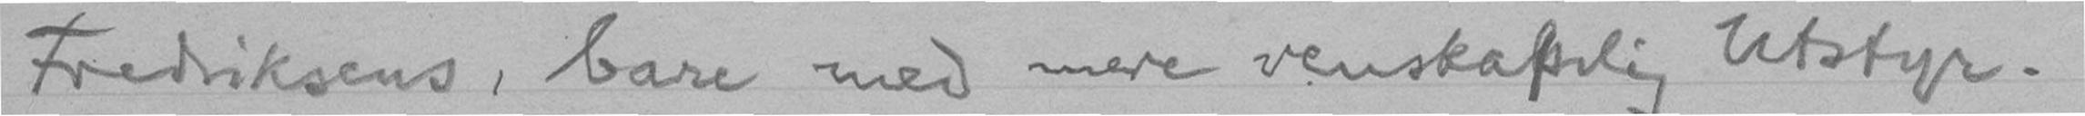Fredriksens, bare med mere venskapelig Utstyr.
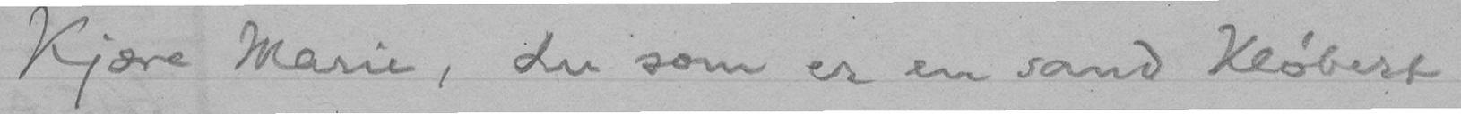Kjære Marie, du som er en Sand Kløbert
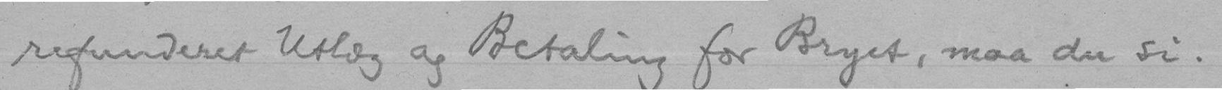refunderet Utlæg og Betaling for Bryet, maa du si.
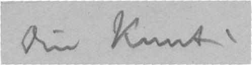Din Knut.
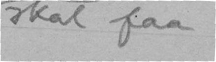skal faa
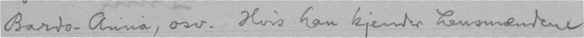Bardo-Anna, osv. Hvis han kjender Lensmændene
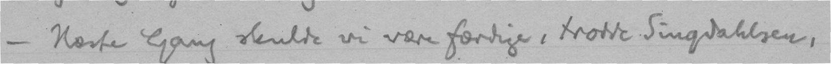- Næste Gang skulde vi være færdige, trodde Singdahlsen,
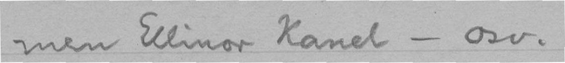men Ellinor Kanel - osv.
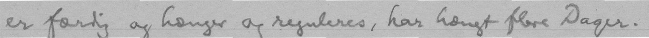er færdig og hænger og reguleres, har hængt flere Dager.
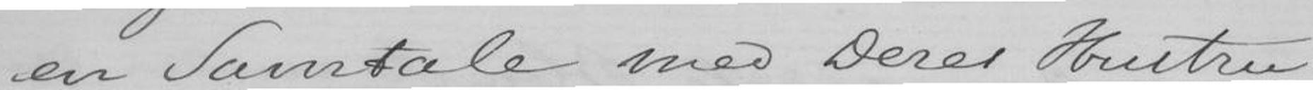en Samtale med Deres Hustru
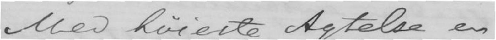Med høieste Agtelse en
